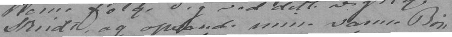Skridt, og opsende min varme Bøn-
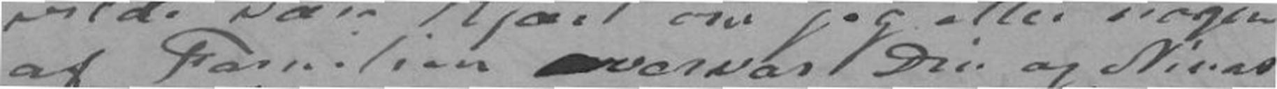af Familien overvar Din og Ninas
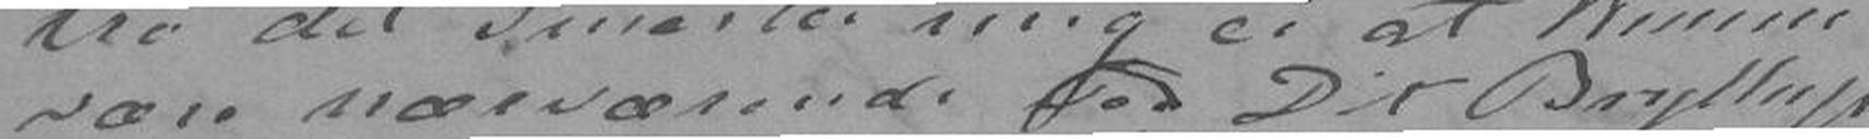være nærværende ved Dit Bryllup,
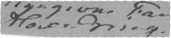Alexr. Grieg.
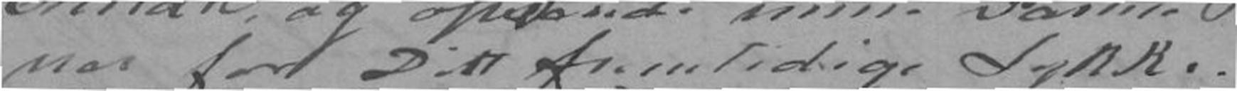ner for Ditt fremtidige Lykke.
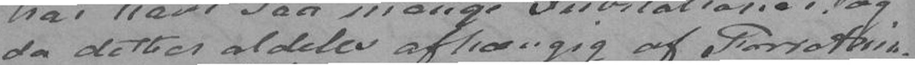da dette er aldeles afhængig af Forretnin-
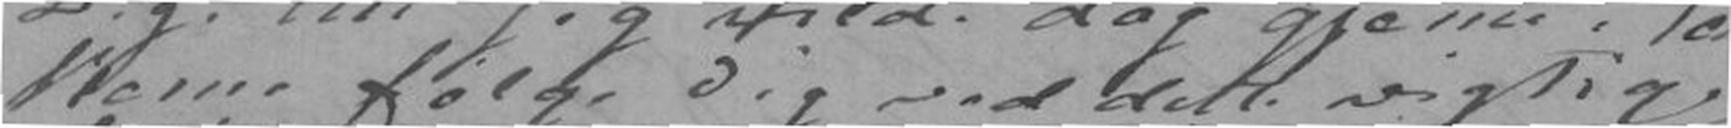kerne følge Dig ved dette vigtige
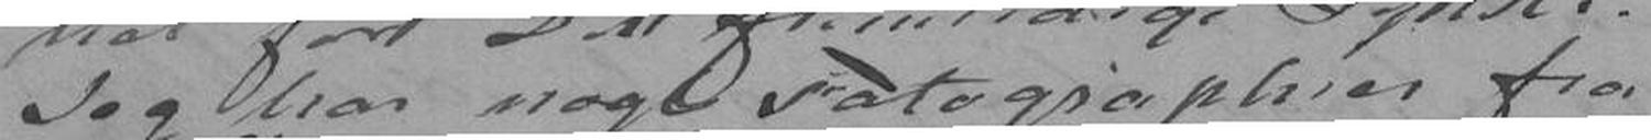Jeg har nogle Fotographier fra
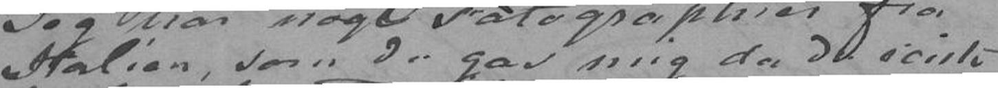Italien, som Du gav mig da Du reiste
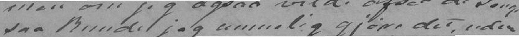saa kunde jeg umuelig gjøre det, uden
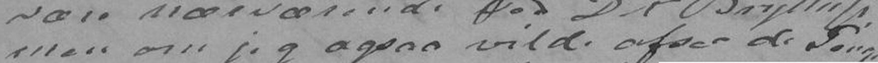men om jeg ogsaa vilde afsee de Penge,
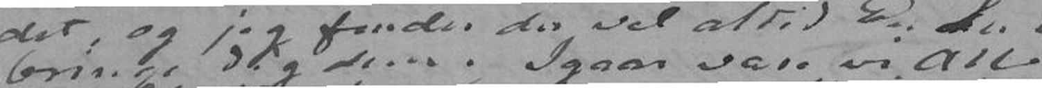bringe Dig dem. Igaar vare vi Alle
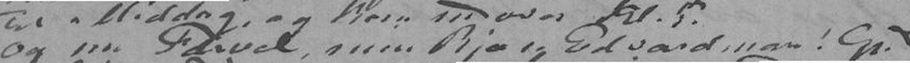Og nu Farvel, min kjære Edvardman! Gud
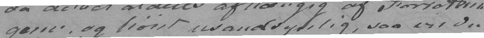gerne, og høist usandsynlig, saa vil Du
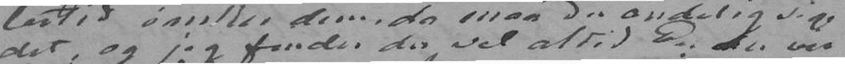det, og jeg finder da vel altid En der vil
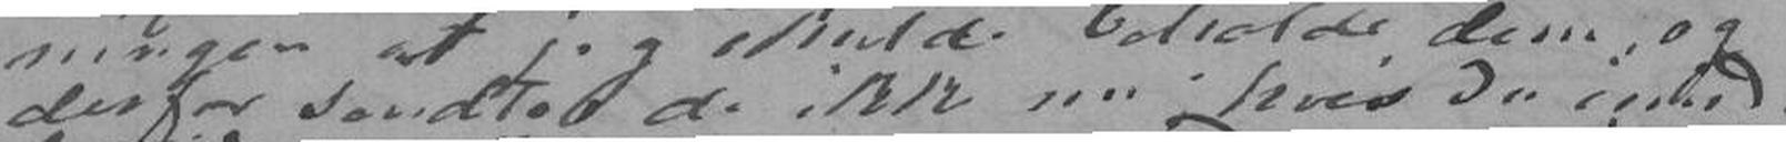derfor sendtes de ikke nu; hvis Du imid-
